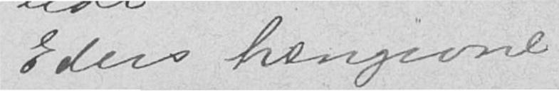Eders hengivne
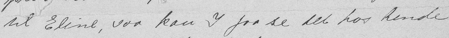til Eline, saa kan I faa se det hos hende.
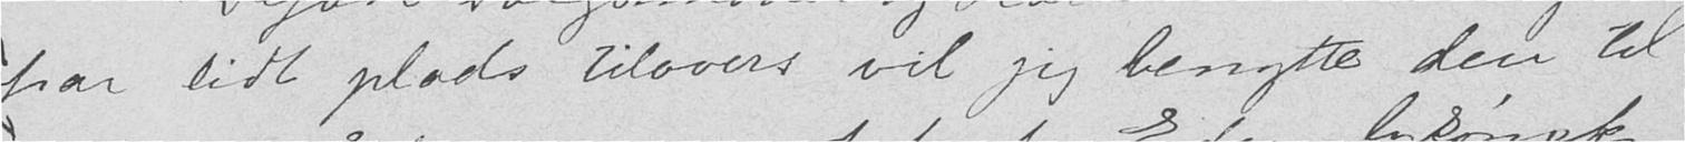har lidt plads tilovers vil jeg benytte den til
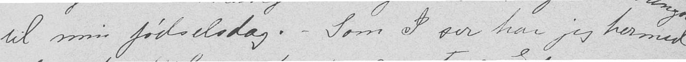til min fødselsdag. – Som I ser har jeg hermed
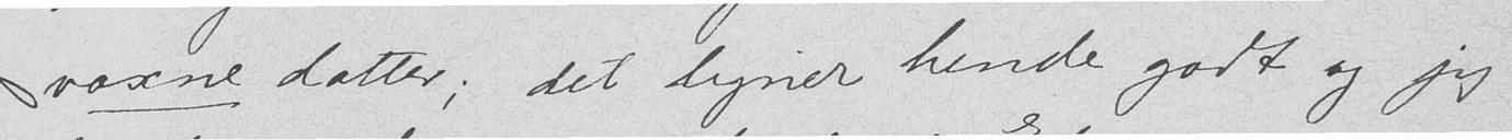voxne datter; det ligner hende godt og jeg
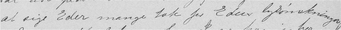at sige Eder mange tak for Eders lykønskninger
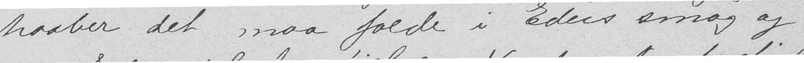haaber det maa falde i Eders smag og
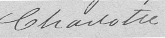Charlotte
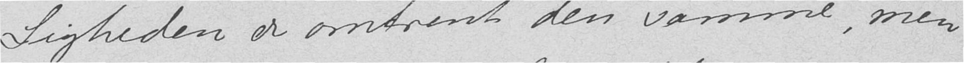Ligheden er omtrent den samme, men
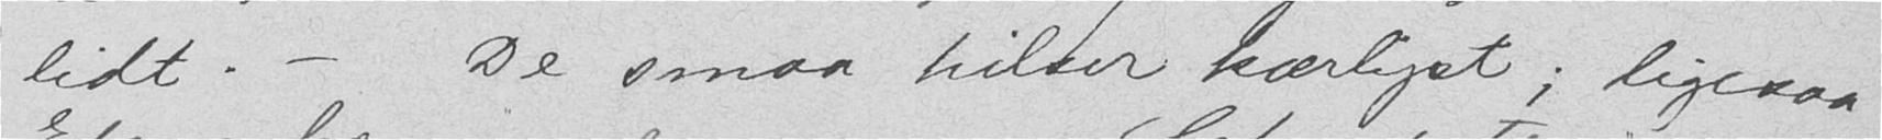lidt. – De smaa hilser kærligst; ligesaa
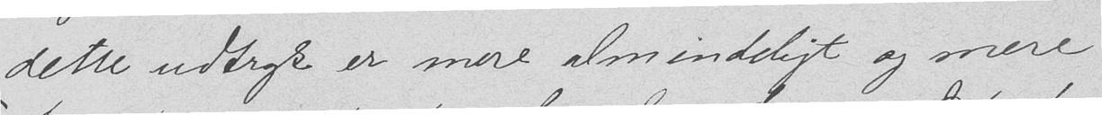dette udtryk er mere almindeligt og mere
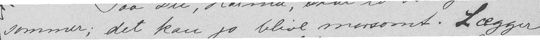sommer; det kan jo blive morsomt. Lægger
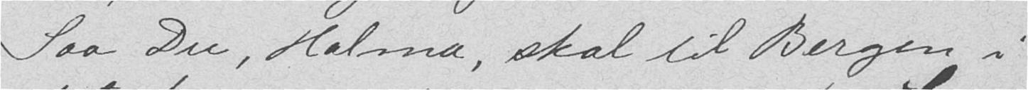Saa Du, Halma, skal til Bergen i
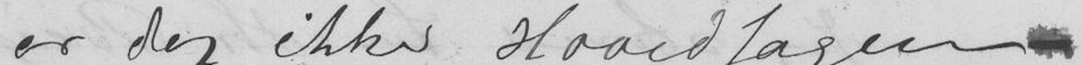er dog ikke Hovedsagen
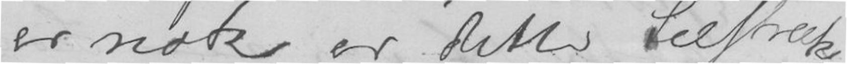er nok er dette tilstræk
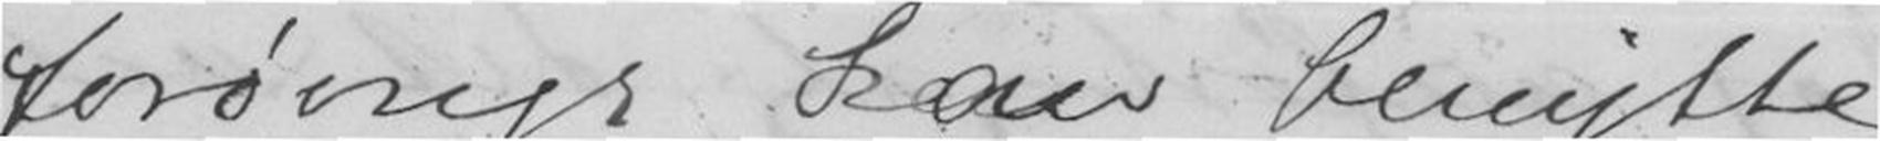forøvrigt kan benytte
